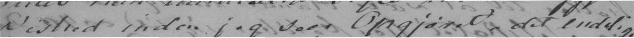vished inden jeg seer Opgjøret, det endelige
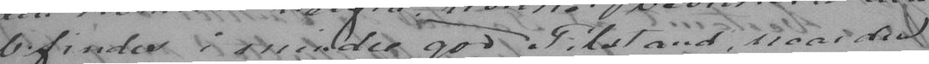befindes i mindre god Tilstand, naar den
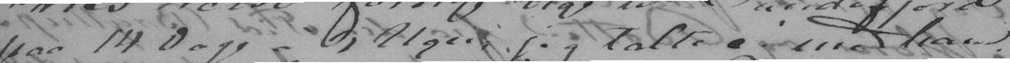paa 14 Dage - 3 Uger, jeg talte ei med ham
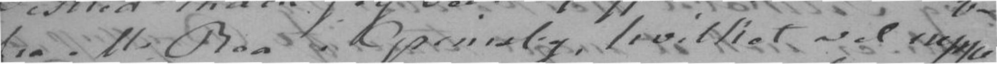fra Mr Rea i Grimsby, hvilket vil neppe
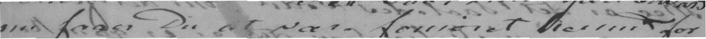nu faaer Du at være fornøiet hermed for
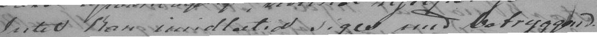Intet kan imidlertid siges med betrygende
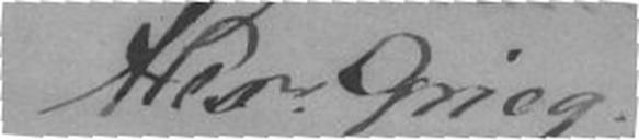Alexr Grieg.
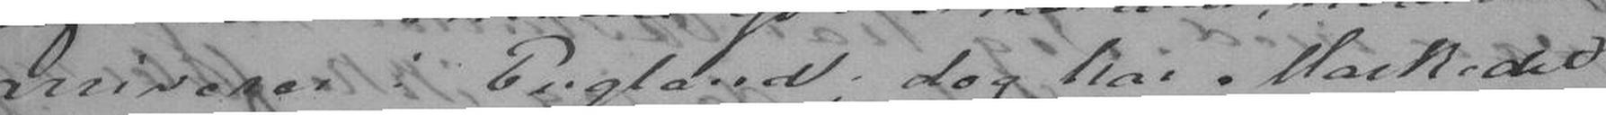arriverer i England, dog kan Markedet
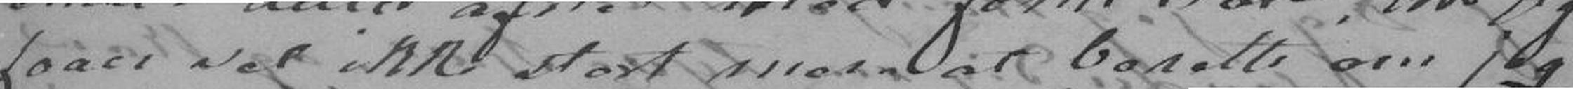faaer vel ikke stort mere at berette om jeg
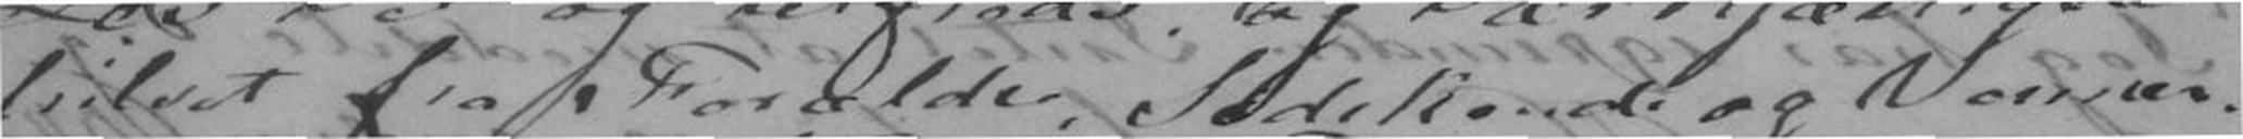hilset fra Forældre, Sødskende og Venner.
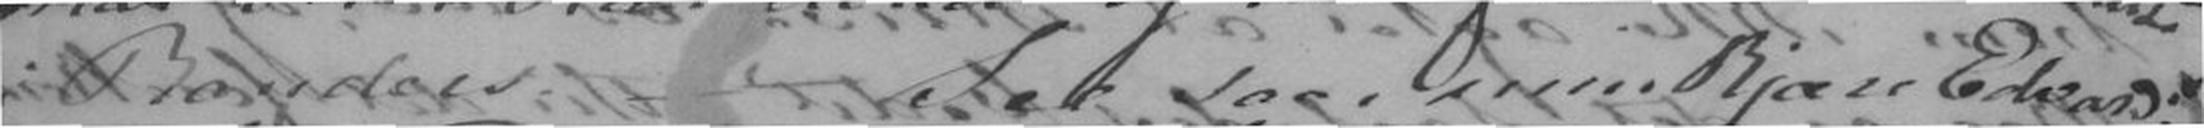i Randers. See saa min kjære Edvard!
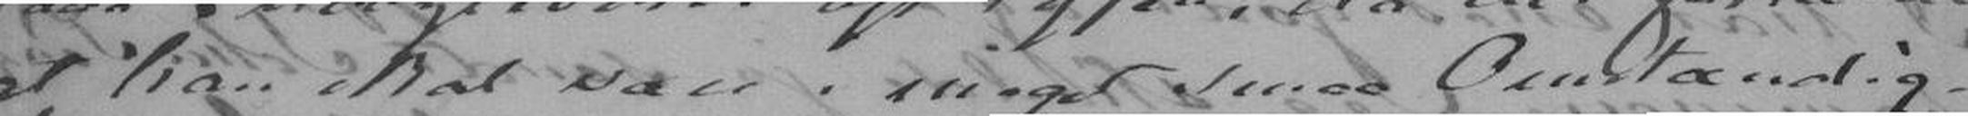at han skal være i meget smaa Omstændig-
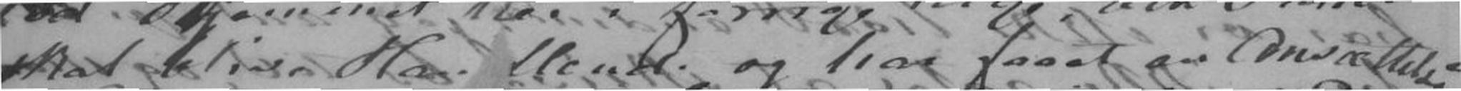skal blive Handlende og har faaet en Ansættelse
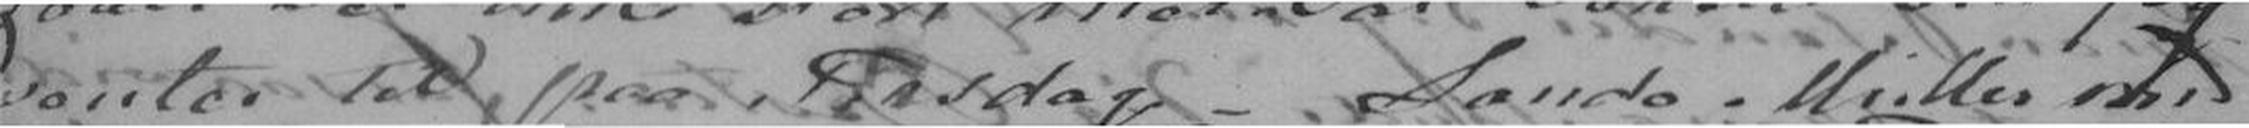venter til paa Tirsdag - Lande Müller med
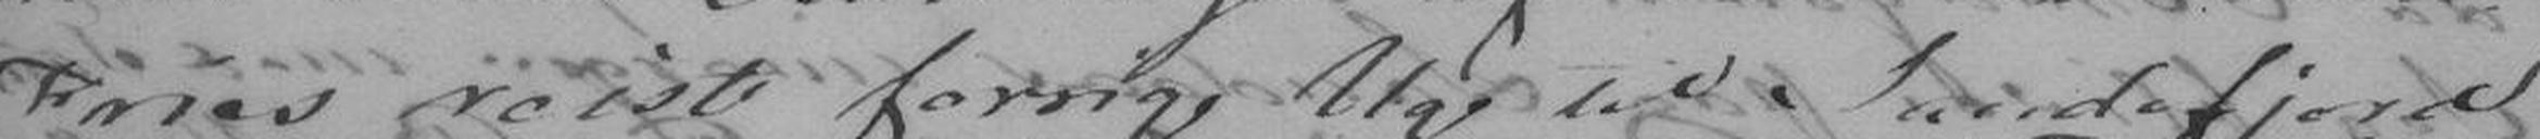Fries reiste forrige Uge til Sandefjord
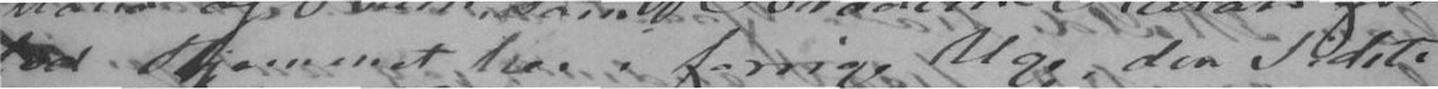lod Hjemmet her i forrige Uge, den sidste
